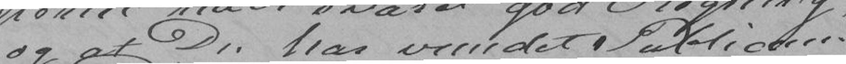og at Du har vundet Publicum
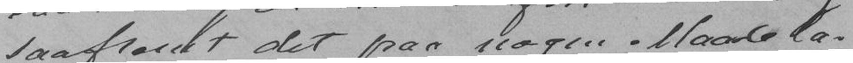saafremt det paa nogen Maade la-
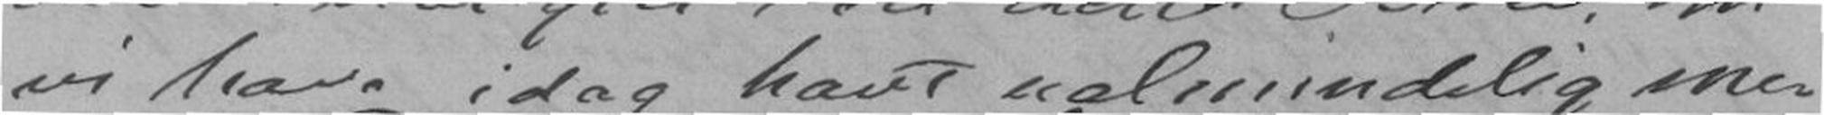vi have idag havt ualmindelig me-
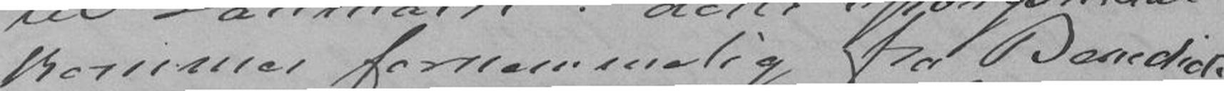kommer fornemmelig fra Benedicte,
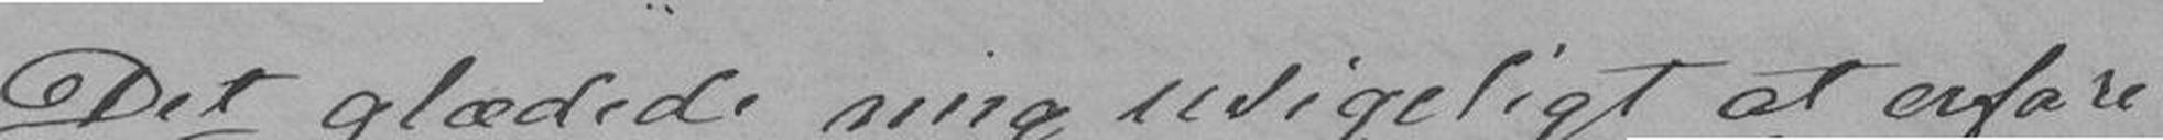Det glædede mig usigelig at erfare
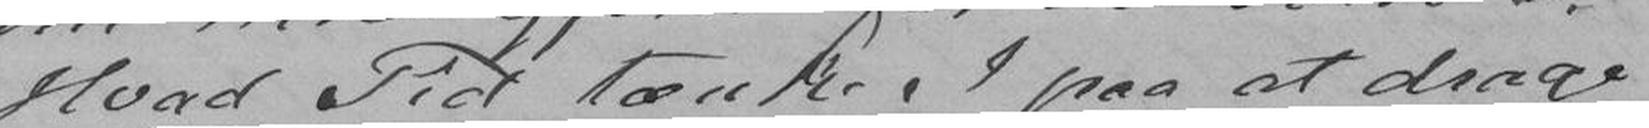Hvad Tid tænkte I paa at drage
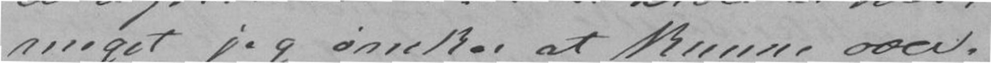meget jeg ønsker at kunne oser
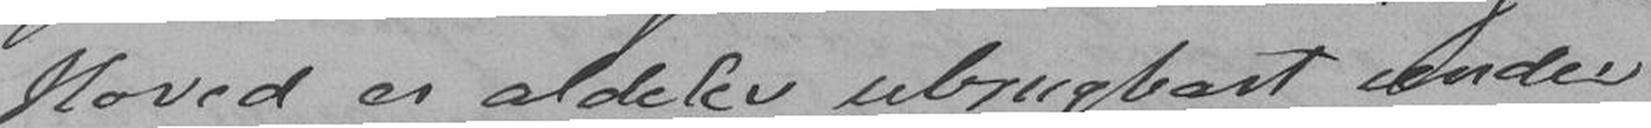Hoved er aldeles ubrugbart under
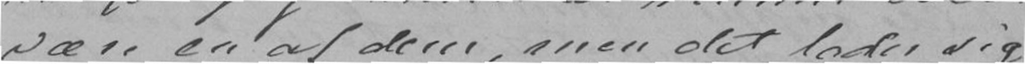være en af dem, men det lader sig
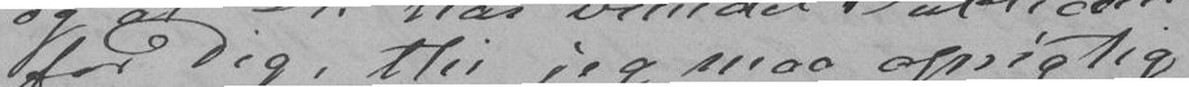for Dig, thi jeg maa oprigtig
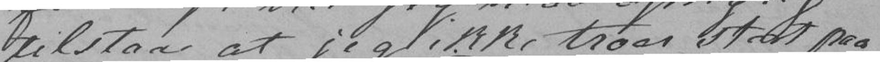tilstaa at jeg ikke troer stort paa
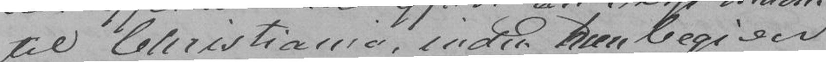til Christiania, inden hun begiver
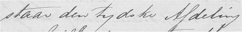staar den tydske Afdeling
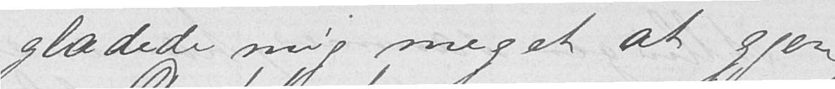glædede mig meget at gjen-
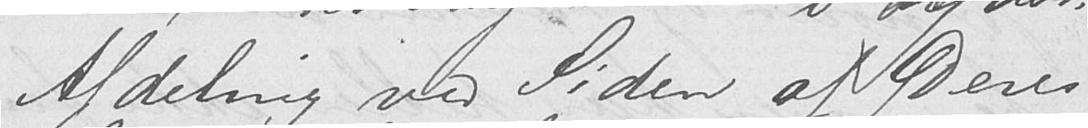Afdeling ved siden af Deres
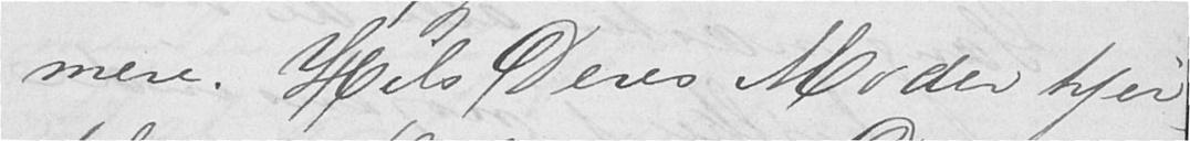mere. Hils Deres Moder hjer-
